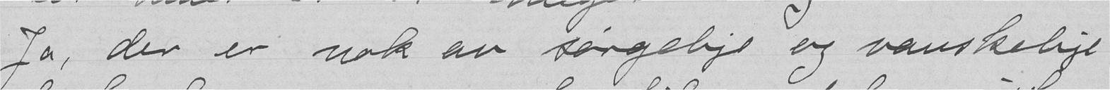Ja, der er nok av sørgelge og vanskelige
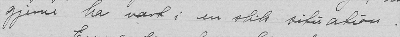gjerne fra vært i en slik situation.
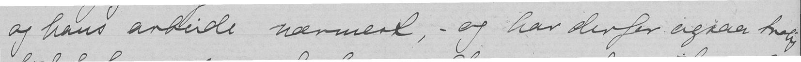og hans arbeide nærmest ,- og har derfor sigsaa trolig
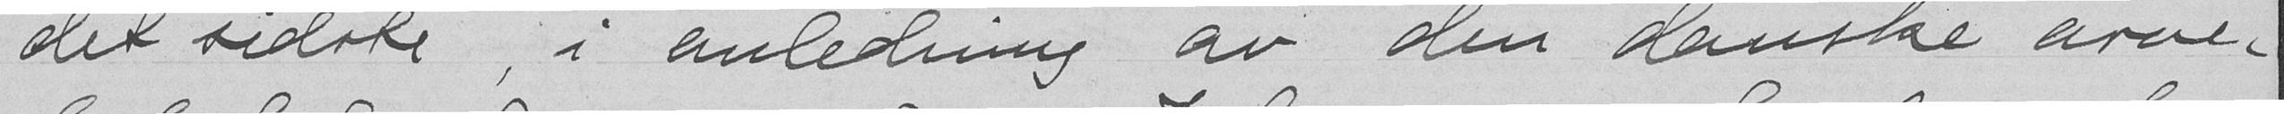det sidste, i anledning ar den danske arve-
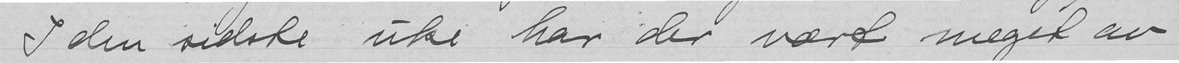I den sidste uke har der være meget av
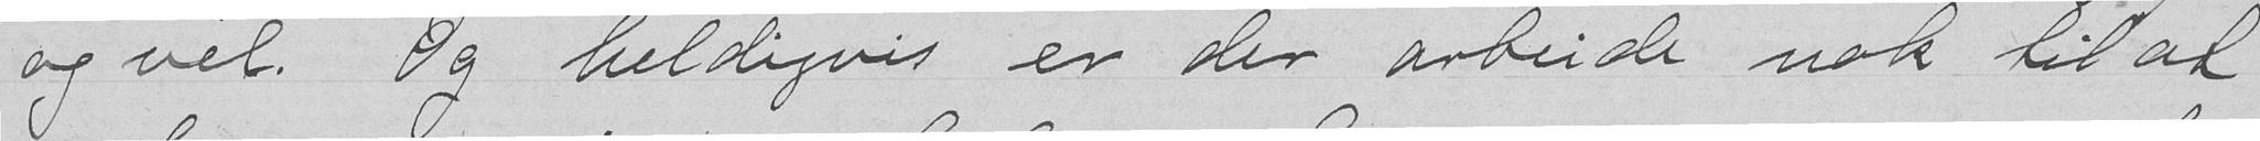og vel. Og heldigvis er der arbeide nok til at
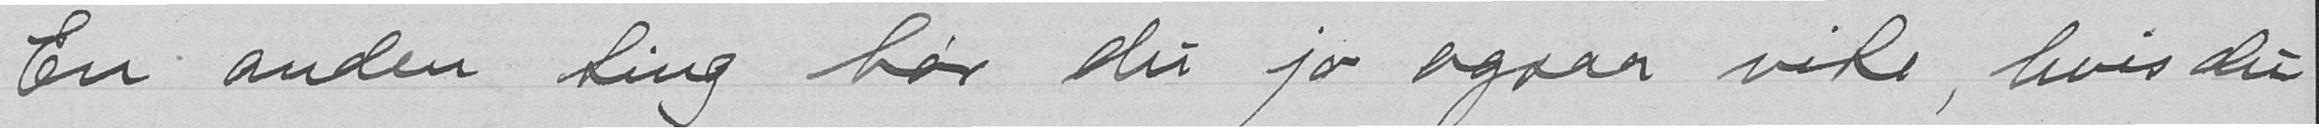En anden ting bør du jo ogsaa vite, hvis du
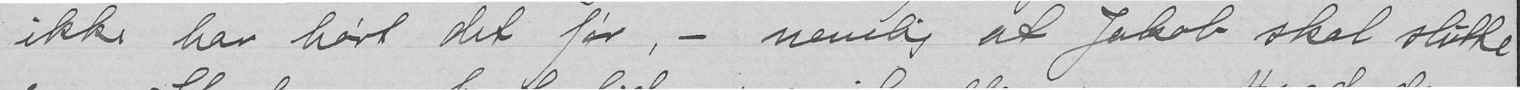ikke har hørt det før, - nemlig at Jakob skal slutte
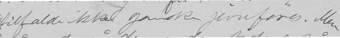tilfælde ikke ganske jevnføres. Men
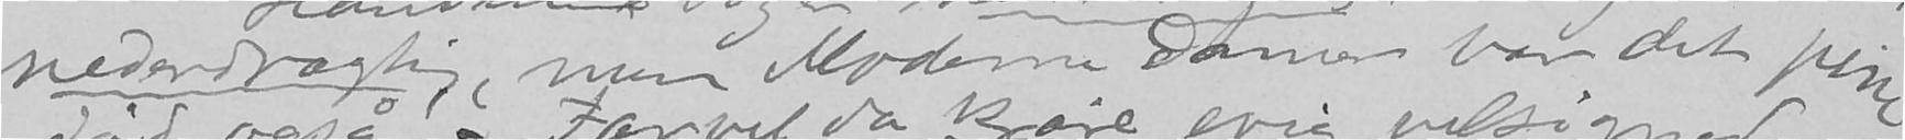nederdrægtig, men Moderne Damer var det pine
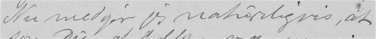Nu medgir jeg naturligvis, at
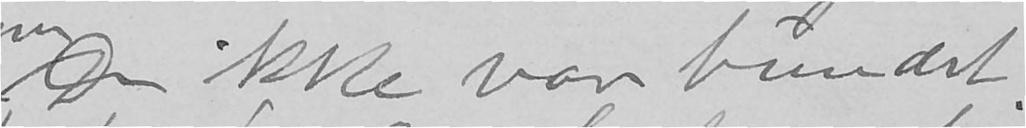Du ikke var bundet
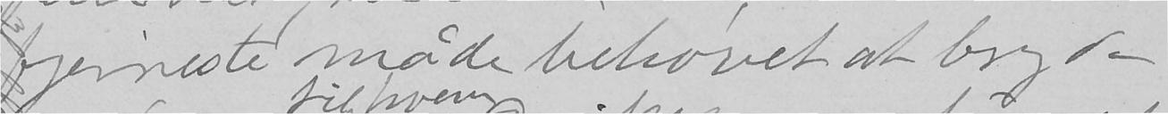fjerneste måde behøvet at bryde
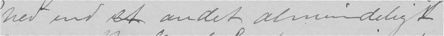hed end et andet almindeligt
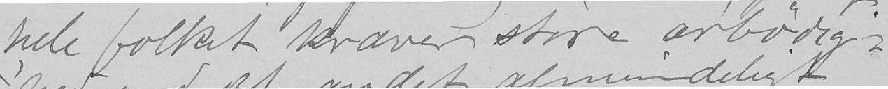hele folket kræver støre arbødig-
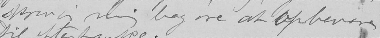skrev jeg mig bag øre at opbevare
